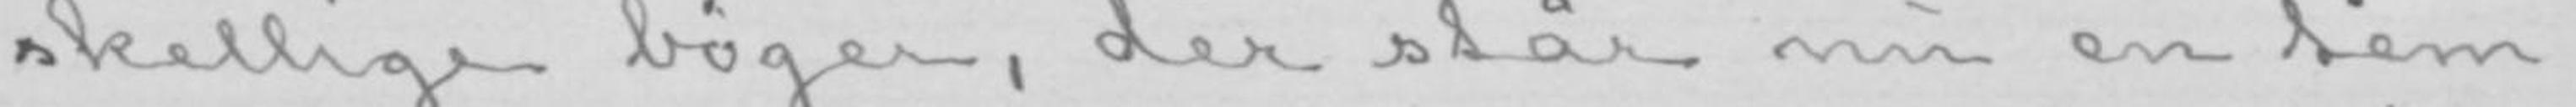skellige bøger, der står nu en tem-
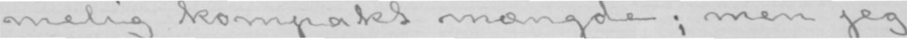melig kompakt mængde; men jeg
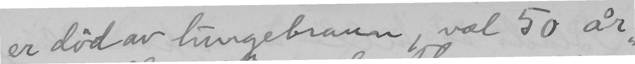er död av lungebrann, væl 50 år
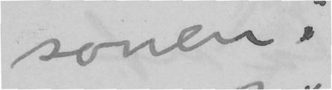sonen!
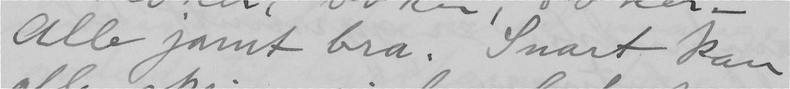alle jamt bra. Snart kan
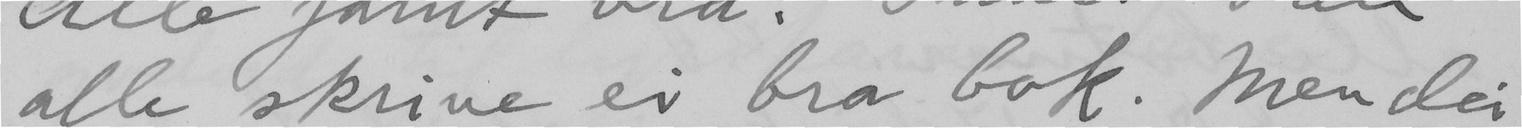alle skrive ei bra bok. Men dei
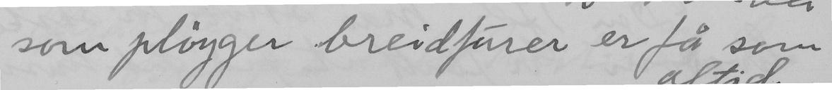som plöyger breidfurer er få som
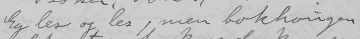Eg les og les, men bokhougen
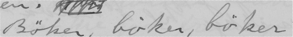Böker, böker, böker!
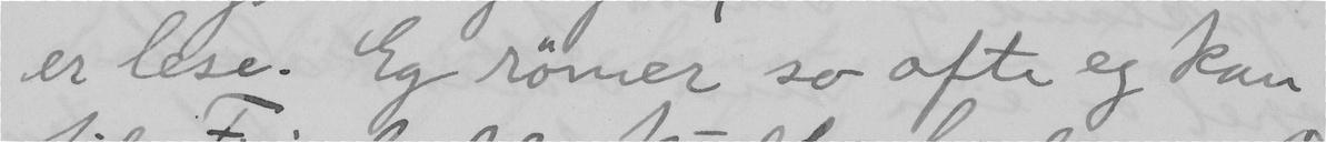er lese. Eg römer so ofte eg kan
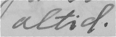altid.
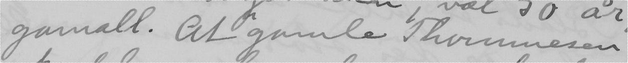gamall. At gamle Thommesen
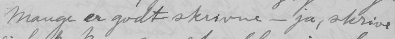Mange er godt skrivne – ja, skrive
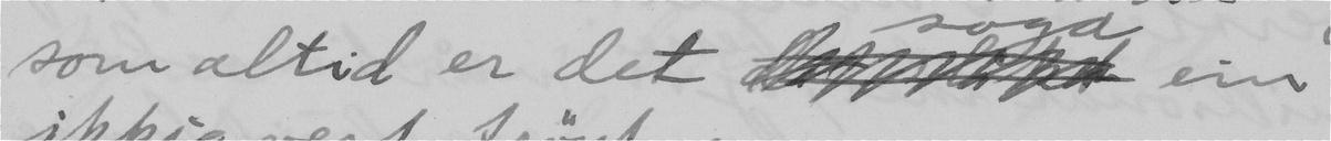som altid er det som altid er det soga ein
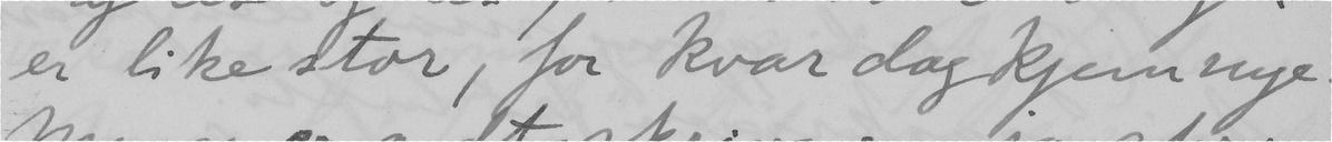er like stor, for kvar dag kjem nye.
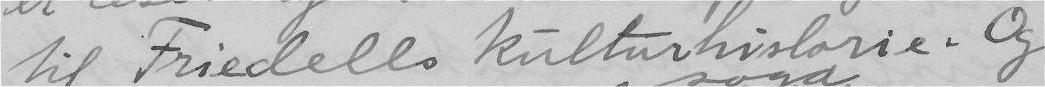til Friedells kulturhistorie! Og
